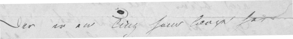Der er en Ting som længe har
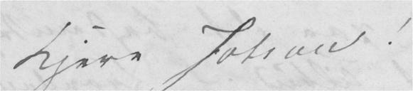Kjere Johan!
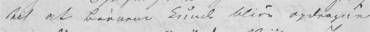til at Børnene kunde blive opdragne
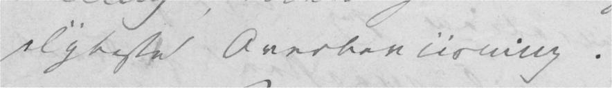dybeste Overbeviisning.
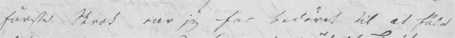første Skræk var jeg for bedøvet til at føle
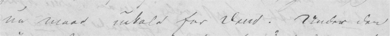nu maae udtale for Dem. Under den
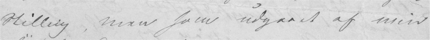Stilling, men som udgaaet af min
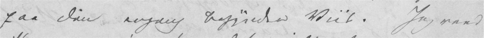paa den engang begyndte Viis. Jeg veed
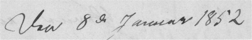Den 8de Januar 1852
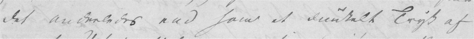det anderledes end som et dunkelt Tryk af
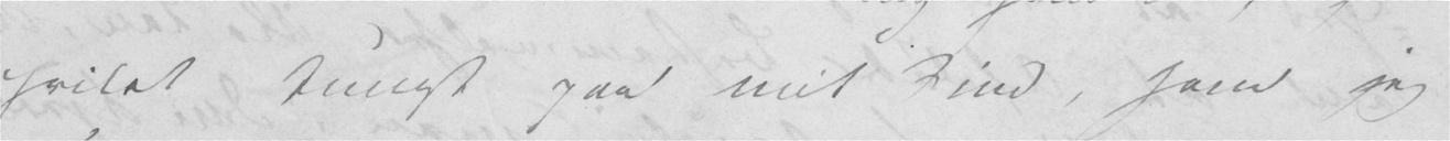hvilet tungt paa mit Sind, som jeg
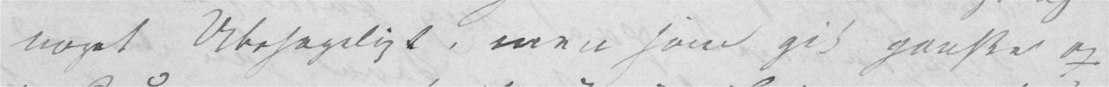noget Ubehageligt, men som gik ganske op
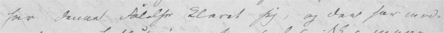har denne Følelse klaret sig, og den har mod-
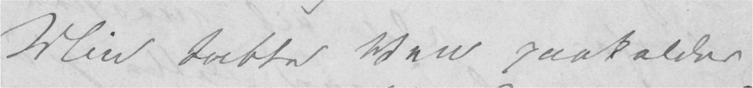Min tabte Ven paakalder
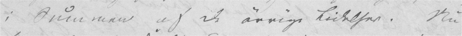i Summen af de øvrige Lidelser. Nu
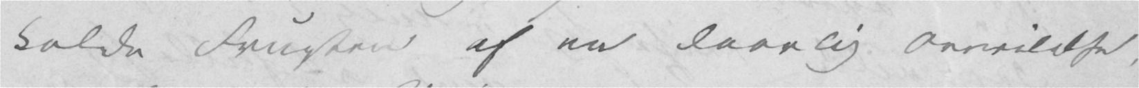kalde Frugten af en daarlig Overilelse,
